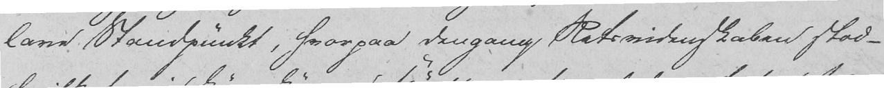lave Standpunkt, hvorpaa dengang Retsvidenskaben stod -
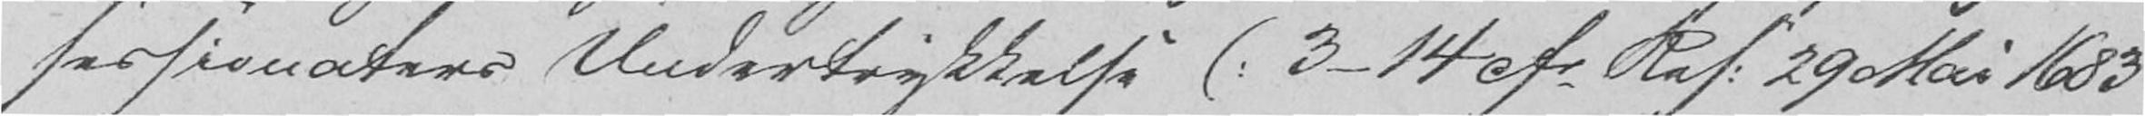sessionaters Undertrykkelse (: 3-14 cfr Res. 29 Mai 1683
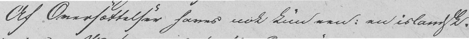Af Oversættelser haves nok kun een: en islandsk.
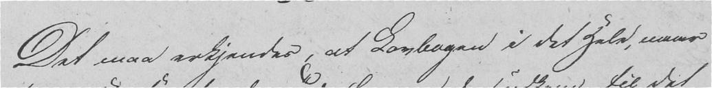Det maa erkjendes, at Lovbogen i det Hele, naar
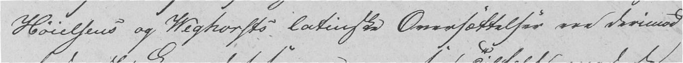Høielsens og Weghorsts latinske Oversættelser ere derimod
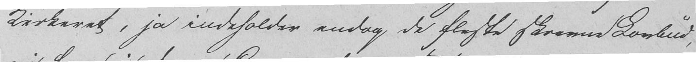Kirkeret, ja indeholder endog de fleste skrevne Lovbud,
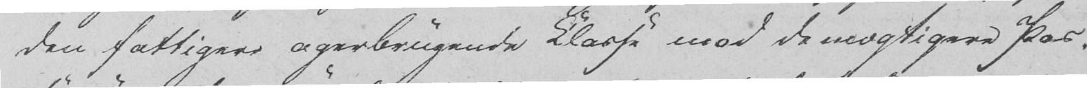den fattigere agerbrugende Klasse mod de mægtigere Pos-
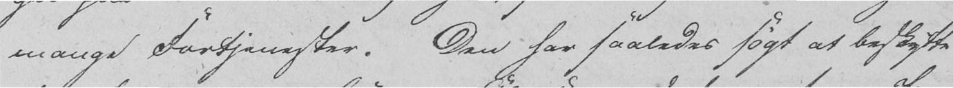mange Fortjenester. Den har saaledes søgt at beskytte
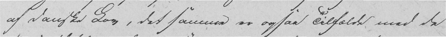af danske Lov, det samme er ogsaa Tilfælde med de
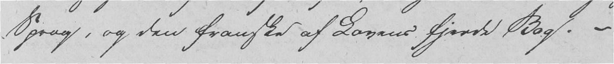Sprog, og den franske af Lovens fjerde Bog. -
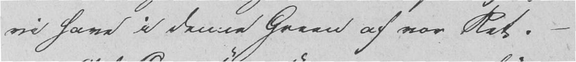vi have i denne Green af vor Ret. -
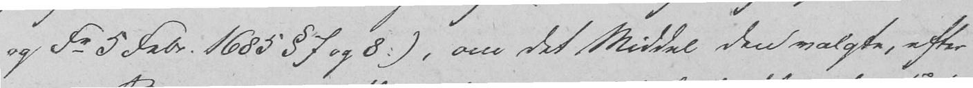og Fr 5 Febr. 1685 § 7 og 8 :), om det Middel den valgte, efter
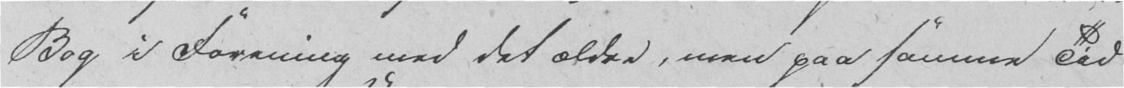Bog i Forening med det ældre, men paa samme Tid
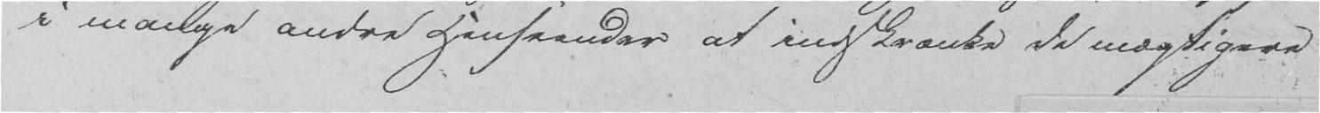i mange andre Henseender at indskrænke de mægtigere
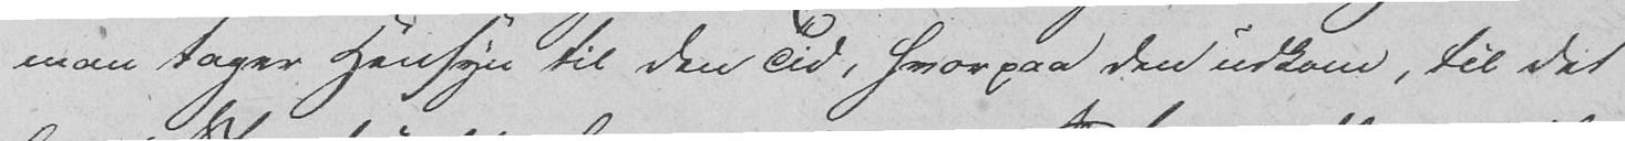man tager Hensyn til den Tid, hvorpaa den udkom, til det
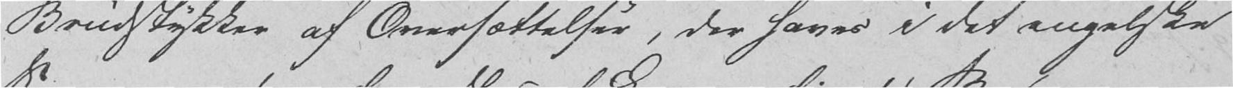Brudstykker af Oversættelser, der haves i det engelske
